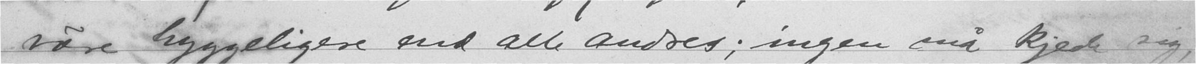være hyggeligere end alle andres; ingen må kjede sig,
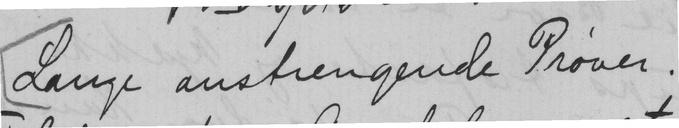Lange anstrengende Prøver.
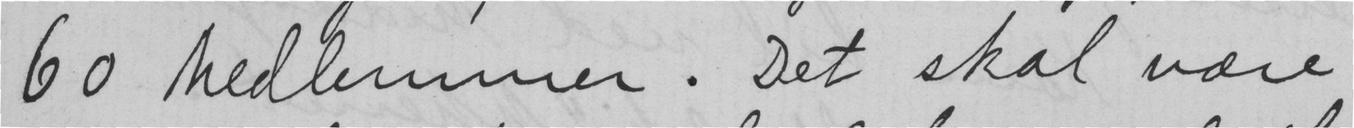60 Medlemmer. Det skal være
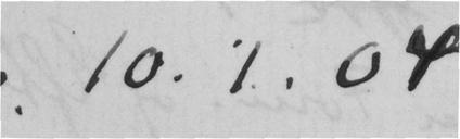10.1.04
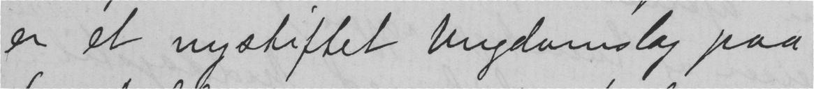er et nystiftet Ungdomslag paa
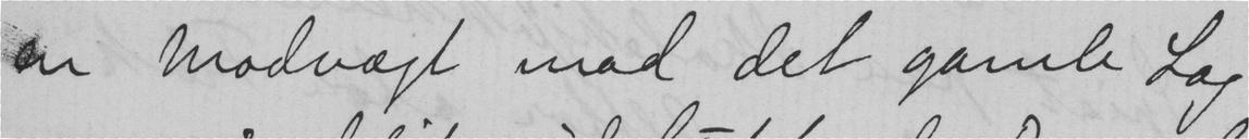en modvægt mod det gamle Lag
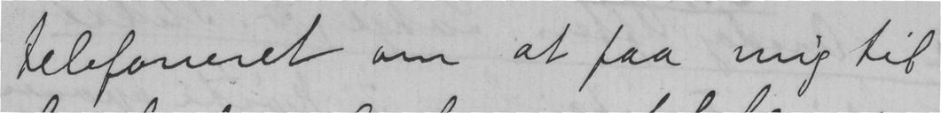telefoneret om at faa mig til
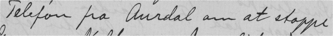Telefon fra Aurdal om at stoppe
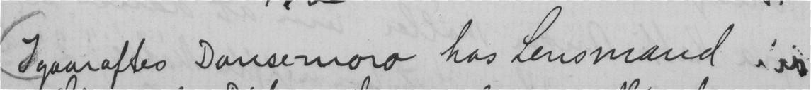Igaaraftes Dansemoro hos Lensmand i
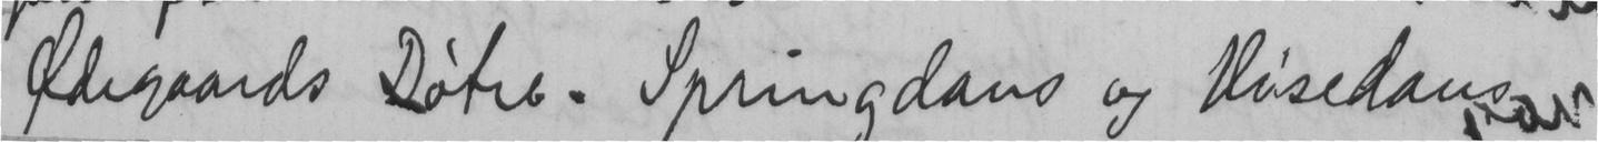Ødegaards Døtre. Springdans og Visedans
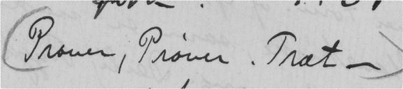(Prøver, Prøver. Træt —
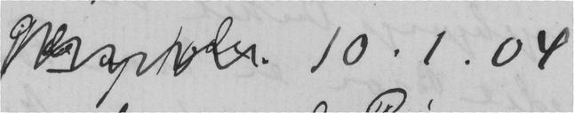. 10.1.04
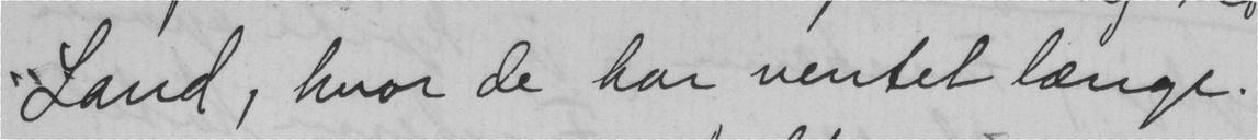Land, hvor de har ventet længe.
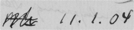11.1.04
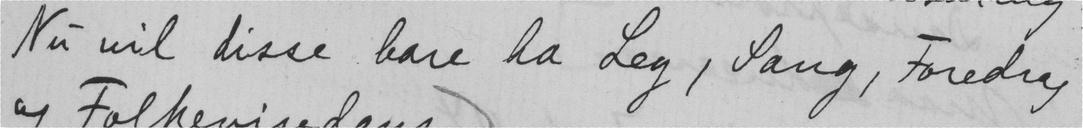Nu vil disse bare ha Leg, Sang, Foredrag
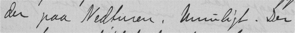der paa Nedturen. Umuligt. Der
